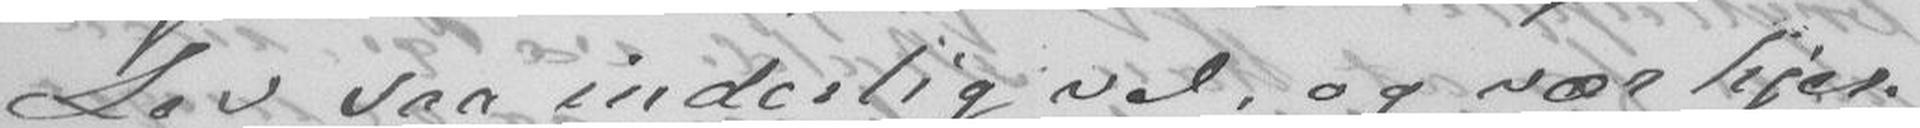Lev saa inderlig vel, og vær hjer-
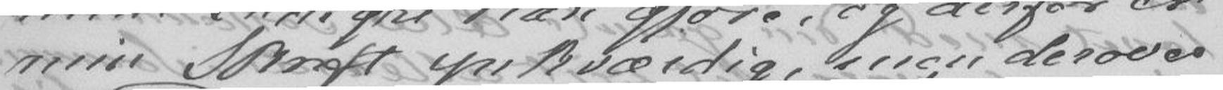min skrift ynkværdig, men derover
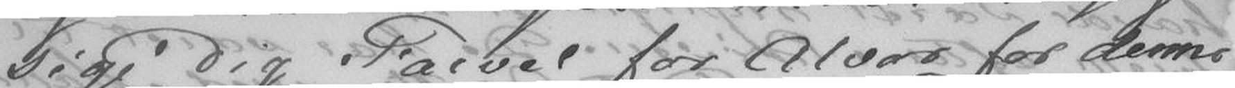sige Dig Farvel for Alvor for denne
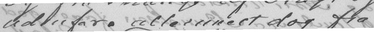udenfor, allermeest fra
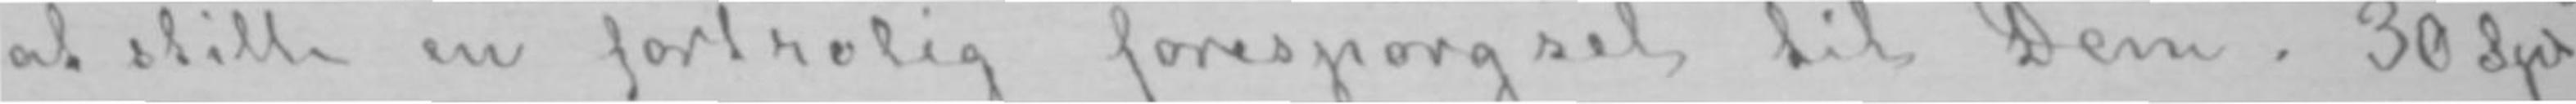at stille en fortrolig forespørgsel til Dem. 30 Spd
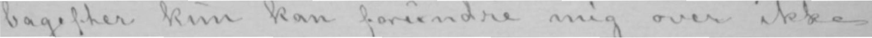bagefter kun kan forundre mig over ikke
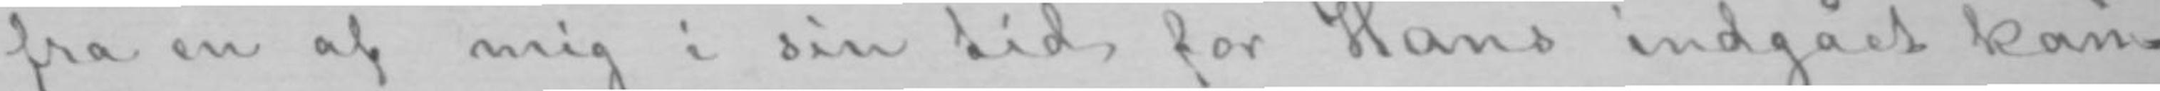fra en af mig i sin tid for Hans indgået kau-
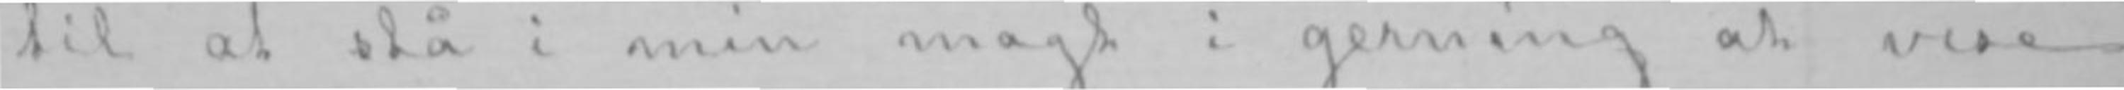til at stå i min magt i gerning at vise
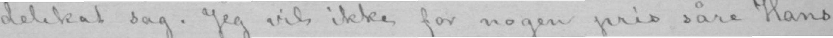delikat sag. Jeg vil ikke for nogen pris såre Hans
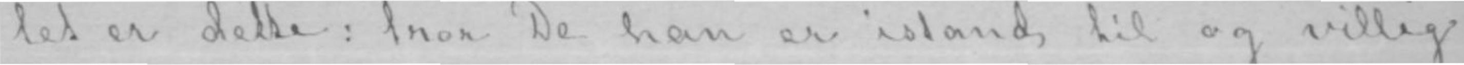let er dette: tror De han er istand til og villig
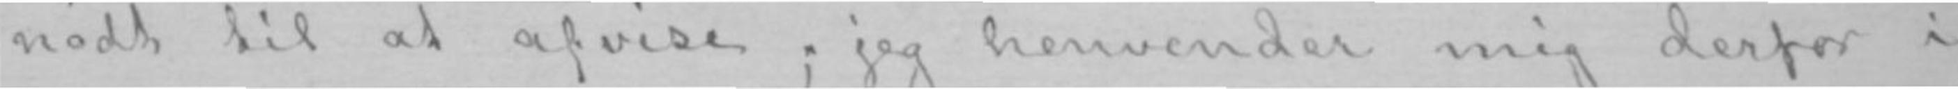nødt til at afvise; jeg henvender mig derfor i
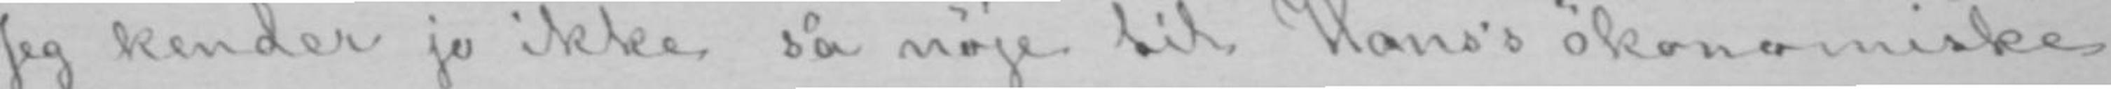Jeg kender jo ikke så nøje til Hans's økonomiske
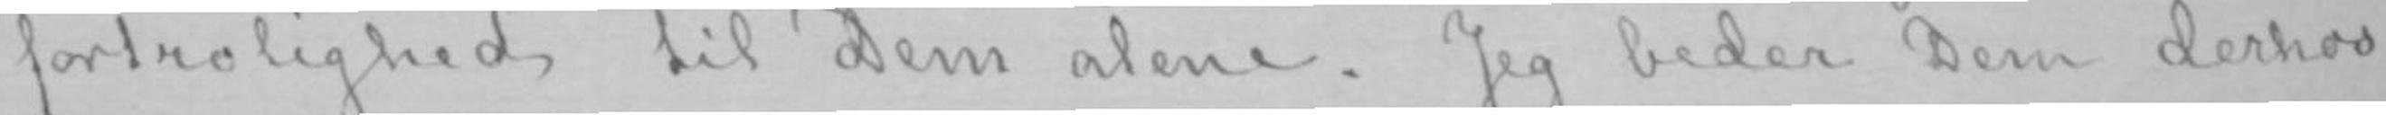fortrolighed til Dem alene. Jeg beder Dem derhos
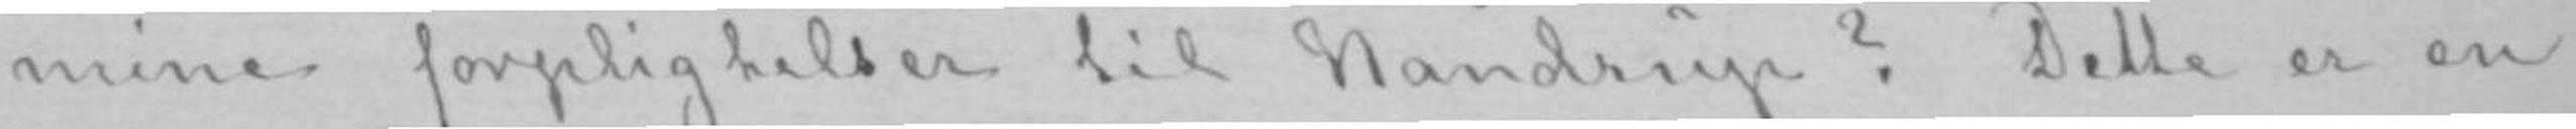mine forpligtelser til Nandrup? Dette er en
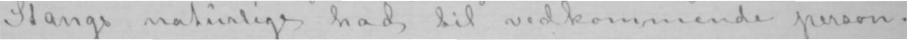Stangs naturlige had til vedkommende person.
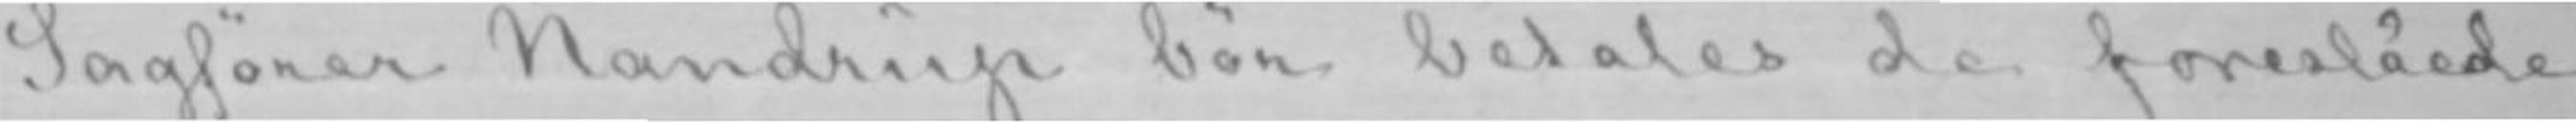Sagfører Nandrup bør betales de foreslåede
